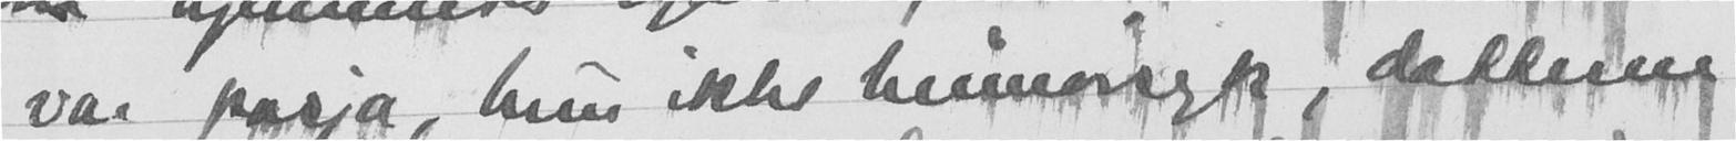var pasja, hun ikke humorisk, datteren
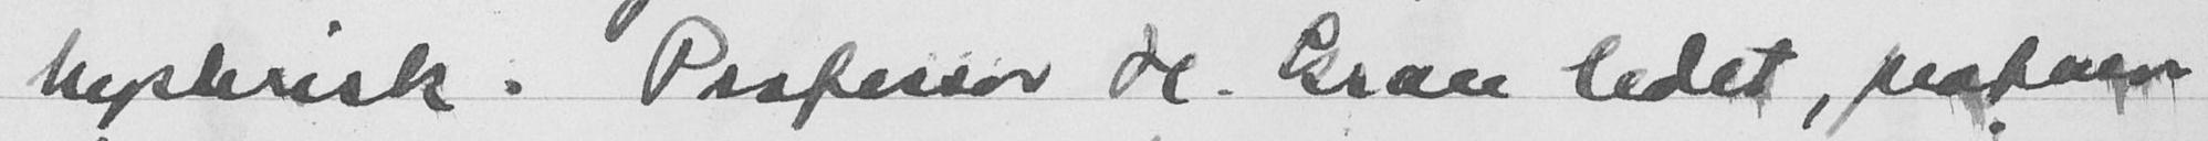hysterisk. Professor H. Gran ledet, professor
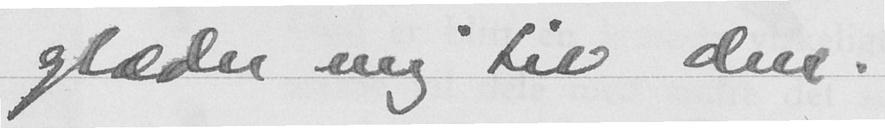glæder mig til den.
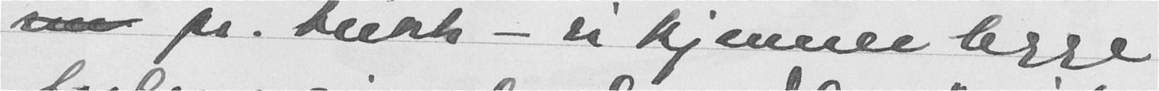pr. blikk - vi kjenner begge
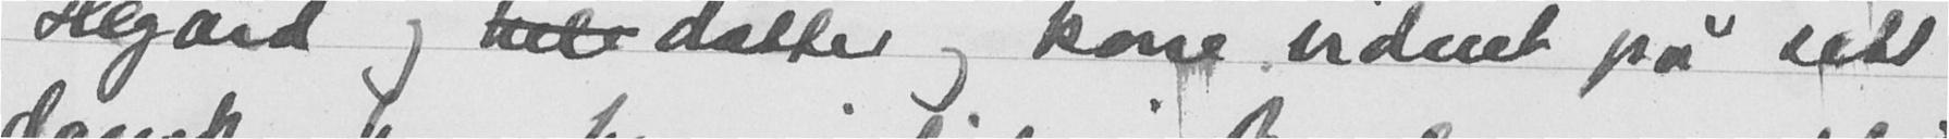Hegård og  datter og kone vidnet på sitt
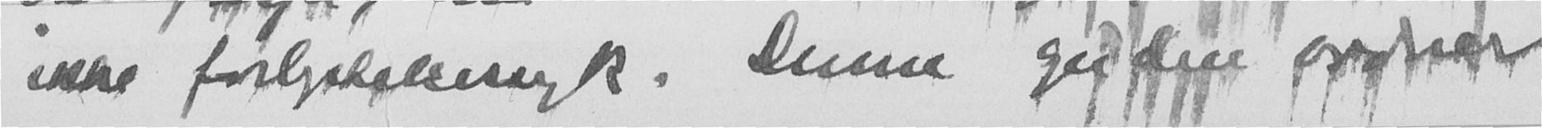ikke forlystelsessyk. Denne guden ordrer
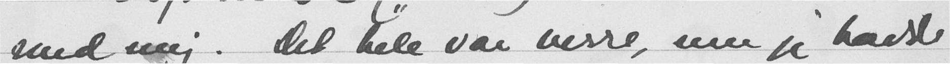med mig. Det hele var verre, enn jeg hadde
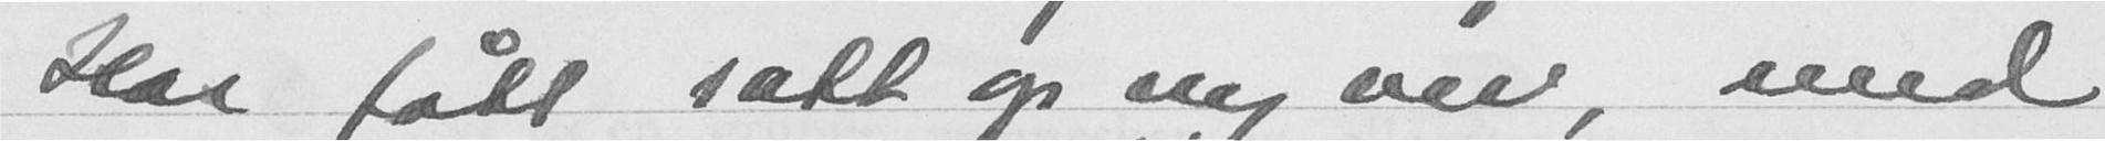Har fått satt op ny vei, med
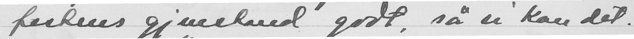festens gjenstand godt, så vi kan det.
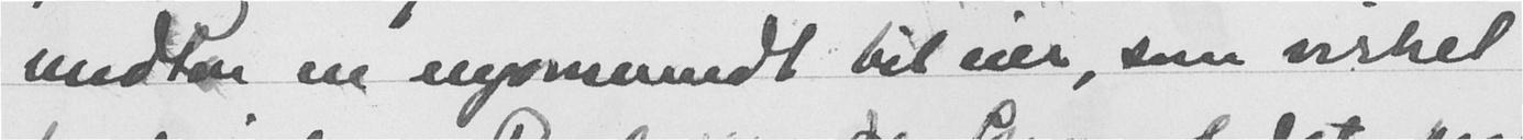medfor en nyomundt til eier, som virket
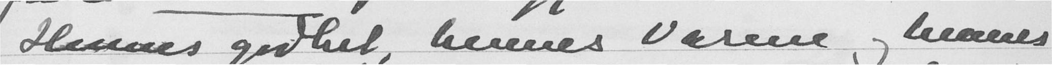Hennes godhet, hennes varme og hennes
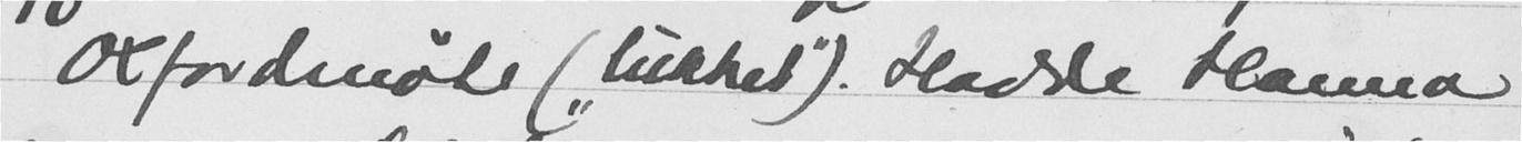Oxfordmøte ("lukket"). Hadde Hanna
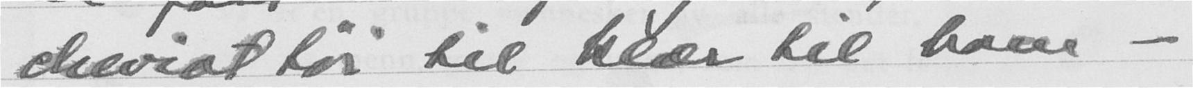shevsat før til klær til ham -
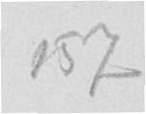157
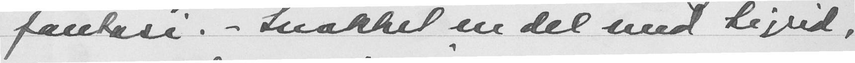fantasi. - Snakket en del med Sigrid,
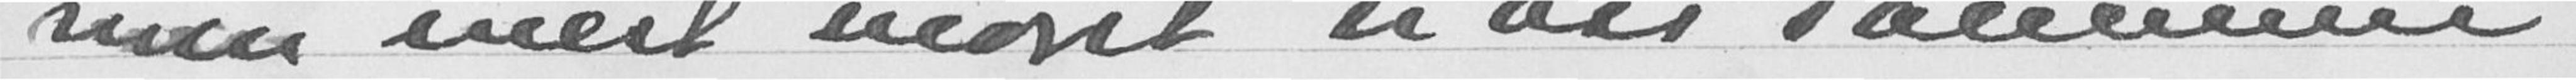men mest moret vi oss sammen
